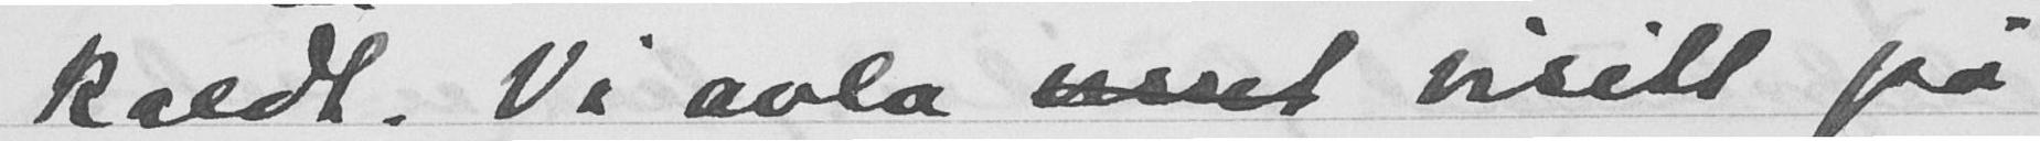koldt. Vi avla  visitt på
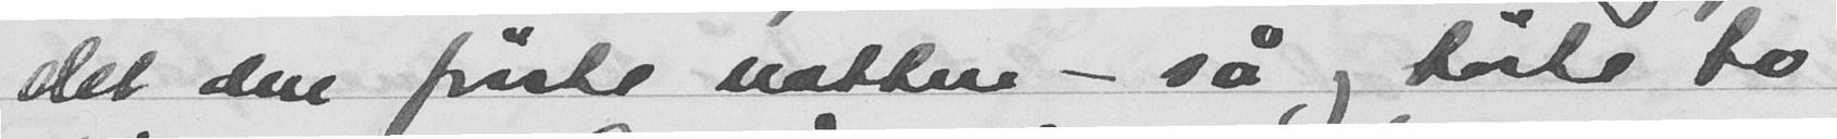det den første natten - så og hørte to
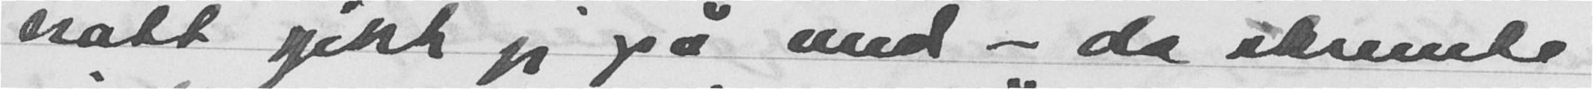natt gikk jeg også med - da skremte
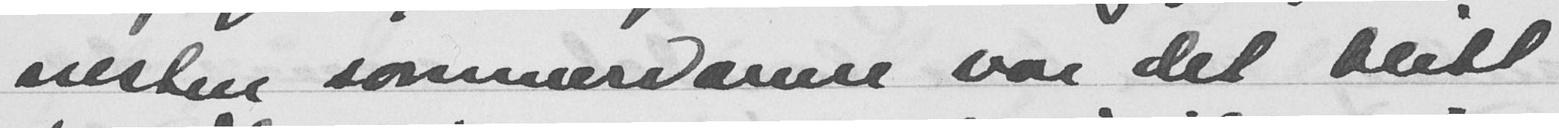nesten sommervarme var det blitt
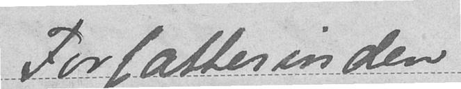Forfatterinden
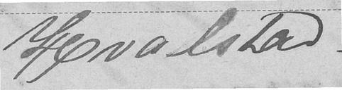Hvalstad.
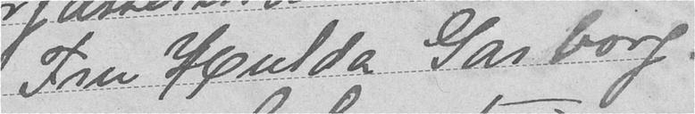Fru Hulda Garborg
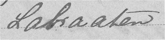Labaaten
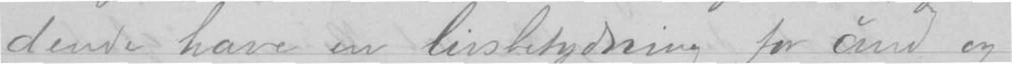dende have en livsbetydning for ånd og
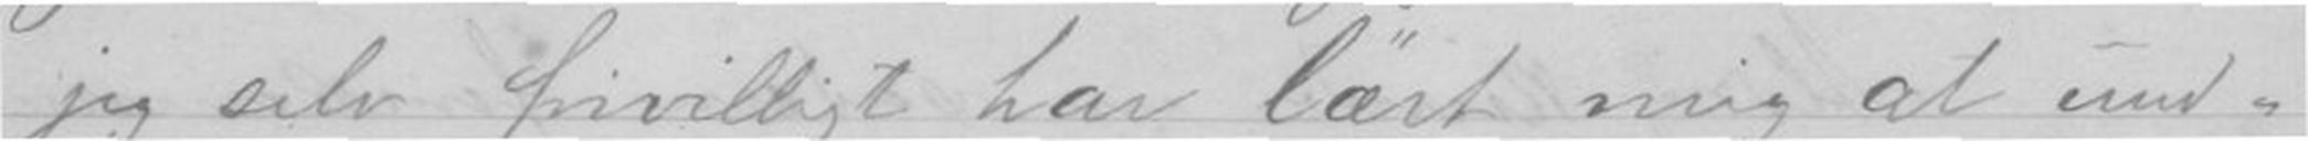jeg selv frivilligt har lärt mig at und-
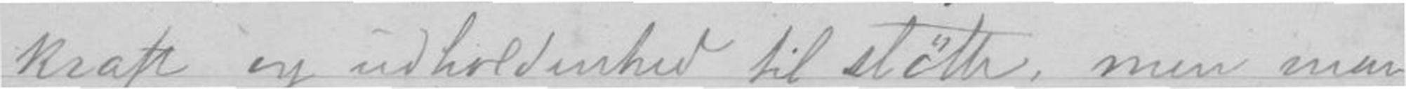kraft og udholdenhed til støtte, men man
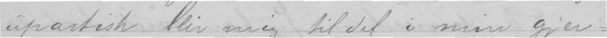upartisk blir mig til del i min gjer-
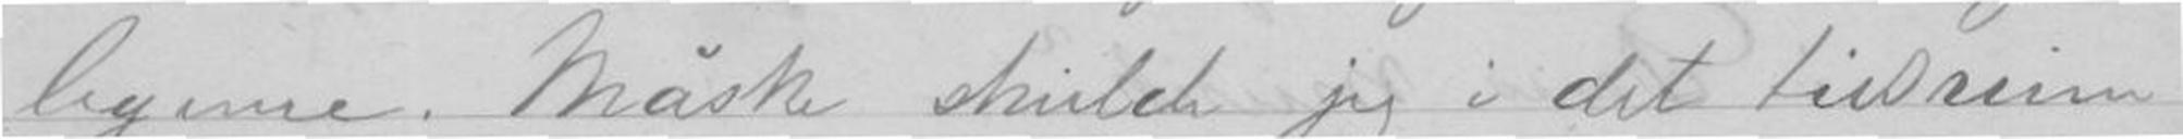legeme. Måske skulde jeg i det tidsrum
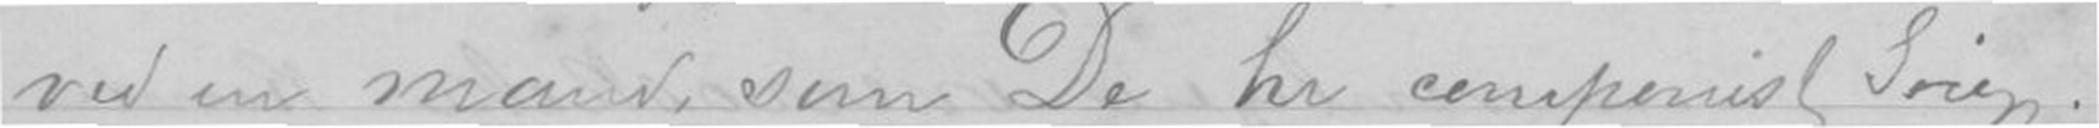ved en mand, som De hr componist Grieg.
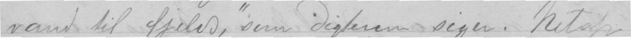vand til fjelds, som Digteren siger. Netop
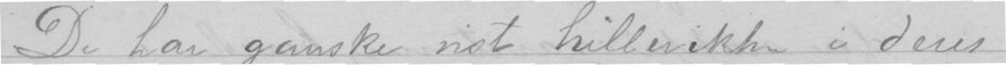De har ganske vist heller ikke i deres
